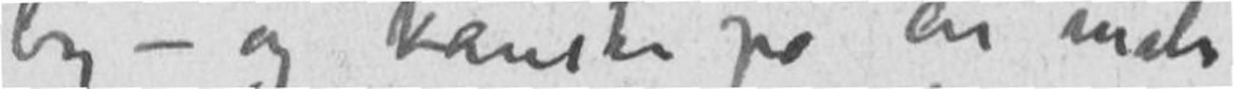by – og kanskje på en måte
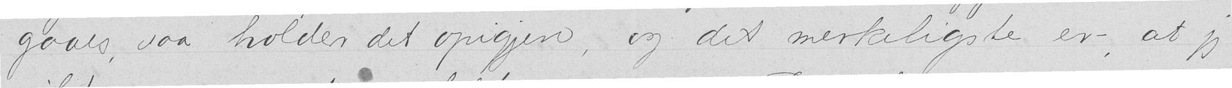gaaes, saa holder det op igjen, og det merkeligste er, at jeg
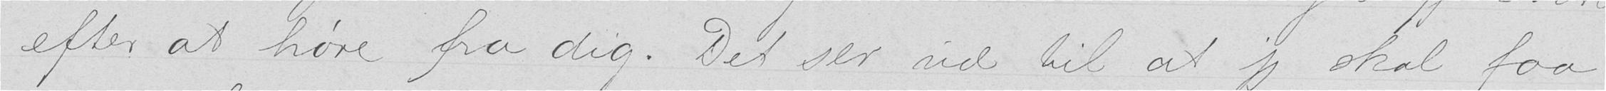efter at høre fra dig. Det ser ud til at jeg skal faa
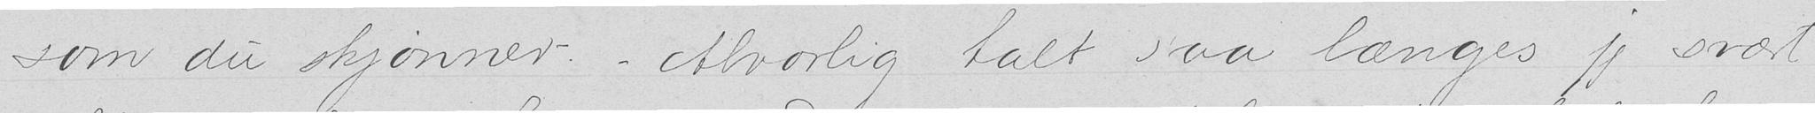som du skjønner. Alvorlig talt saa længes jeg svært
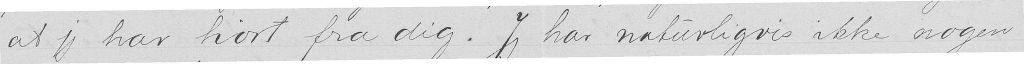at jeg har hørt fra dig. Jeg har naturligvis ikke nogen
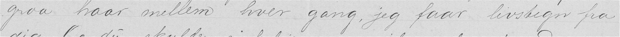graa haar mellem hver gang, jeg faar livstegn fra
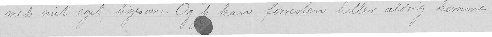med mit eget, ligesom. Og jeg kan forresten heller aldrig komme
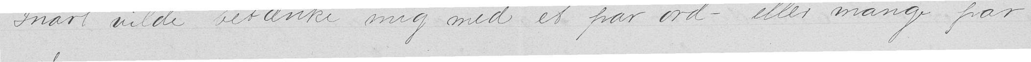snart vilde betænke mig med et par ord - eller mange par
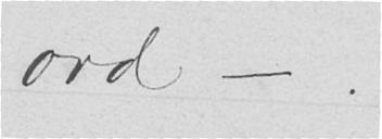ord -.
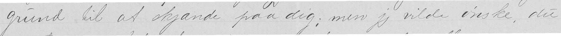grund til at skjænde paa dig; men jeg vilde ønske, du
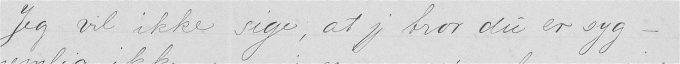Jeg vil ikke sige, at jeg tror du er syg -
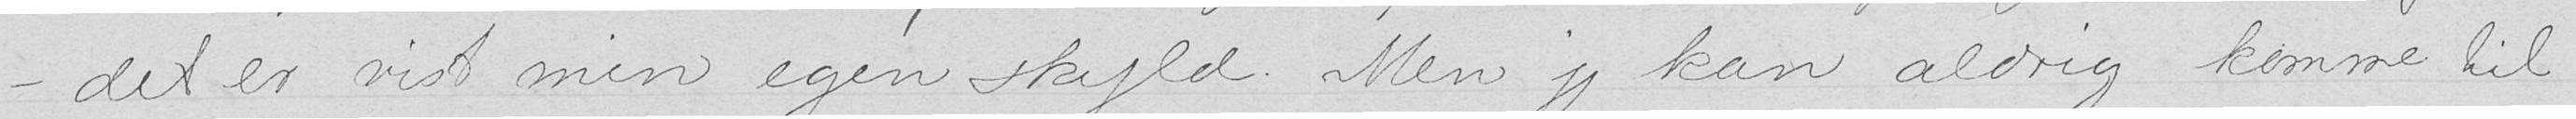- det er vist min egen skyld. Men jeg kan aldrig komme til
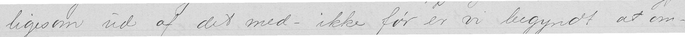ligesom ud af det med - ikke før er vi begyndt at om-
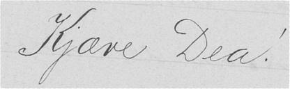Kjære Dea!
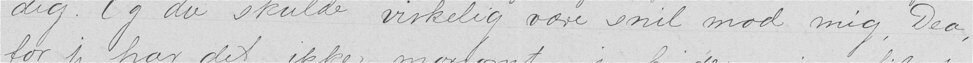dig. Og du skulde virkelig være snil mod mig, Dea,
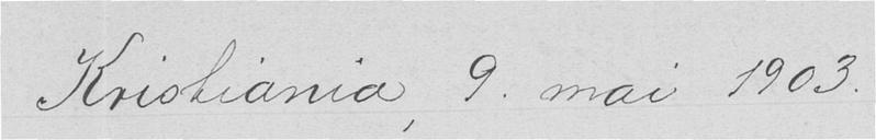Kristiania, 9. mai 1903.
